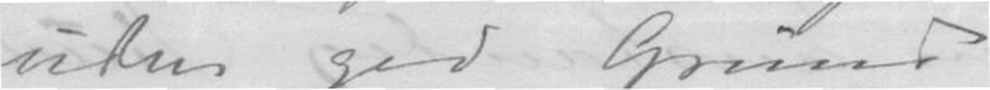uten god Grund
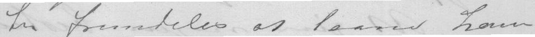til fremdeles at laane ham
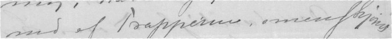ned af Trapperne, omendskjøndt
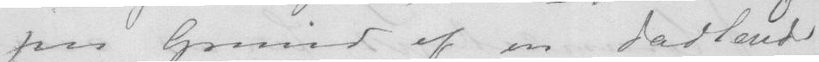paa Grund af en dadlend
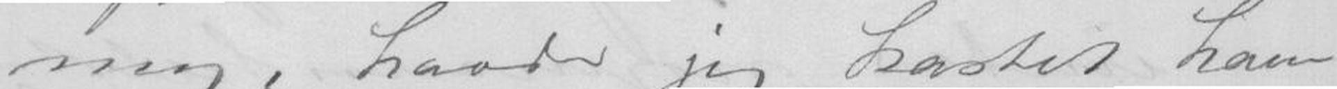mig, havde jeg kastet ham
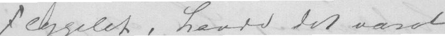Flyglet, havde det været
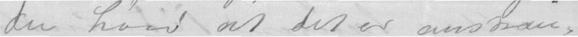du hved at det er anstræn-
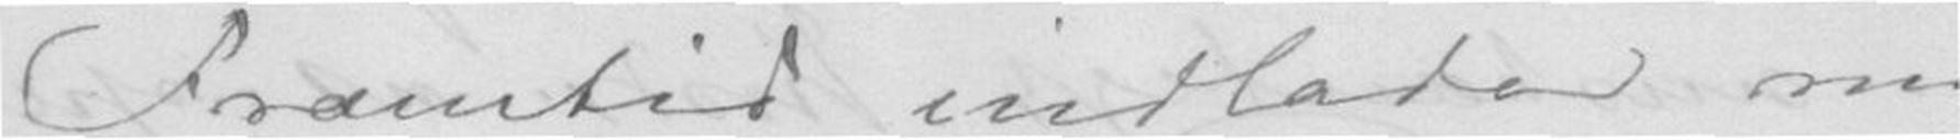Fremtid indlader mig
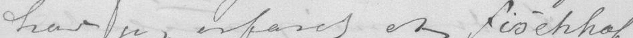har jeg erfaret at Fischof
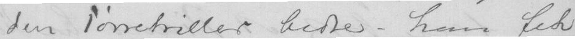den Tønetriller bedre - han fik
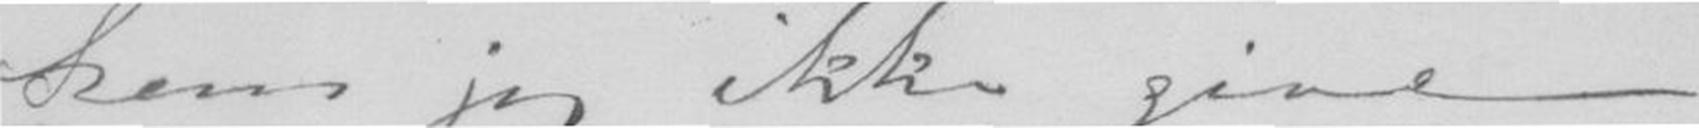kan jeg ikke give
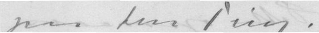paa den Ting.
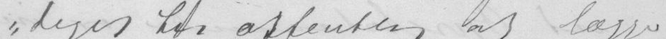diget til offentlig at lægge
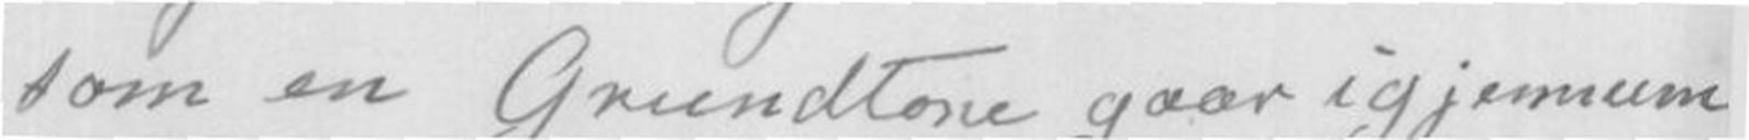som en Grundtone gaar igjennem
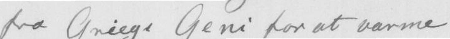fra Griegs Geni for at varme
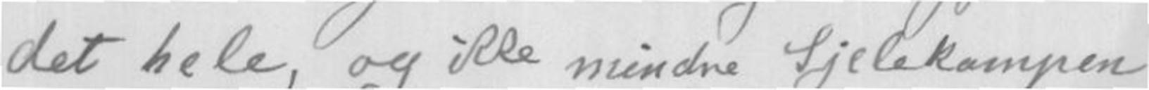det hele, og ikke mindre Sjelekamper
